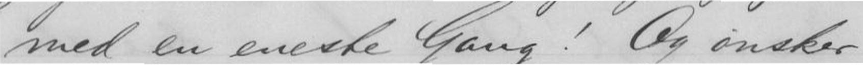med en eneste Gang! Og ønsker
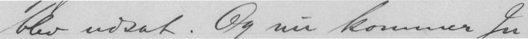blev udsat. Og nu kommer Ju-
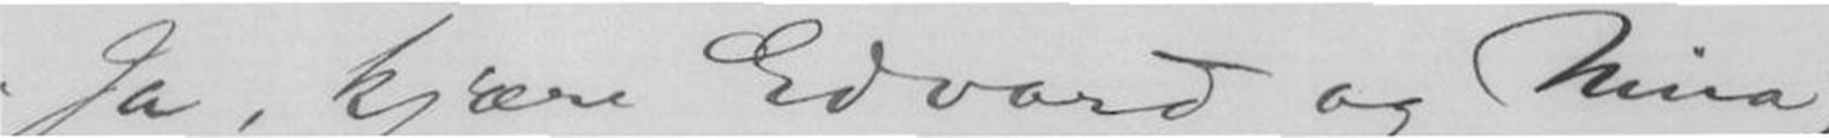Ja, kjære Edvard og Nina,
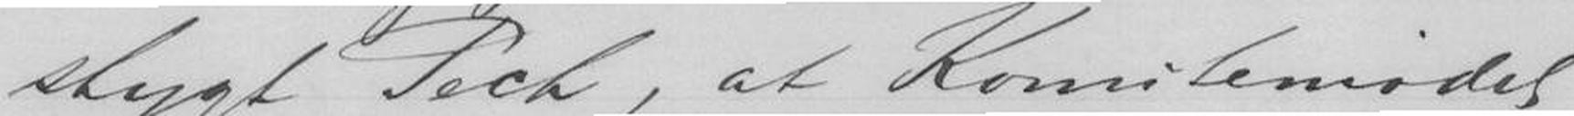stygt Pech, at Komitemødet
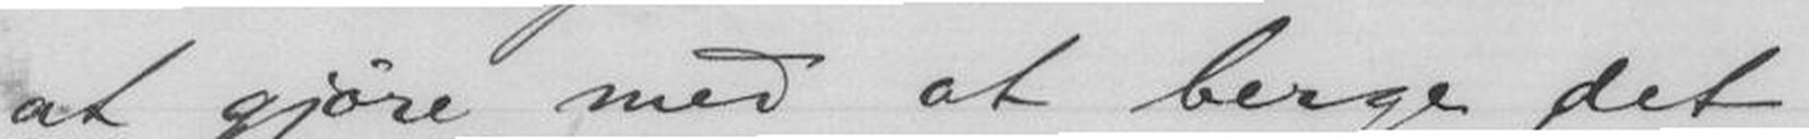at gjøre med at berge det
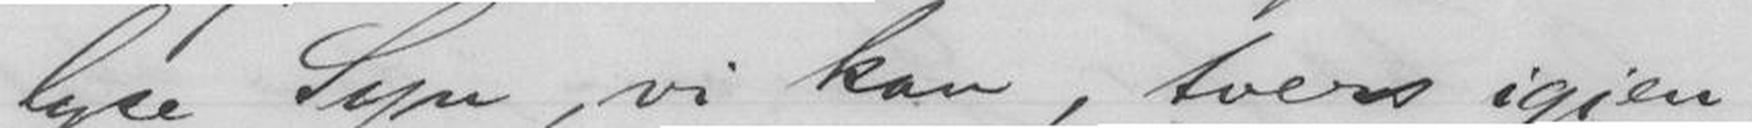lyse Syn, vi kan, tvers igjen-
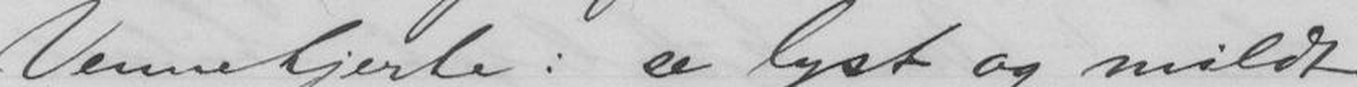Vennehjerte: se lyst og mildt
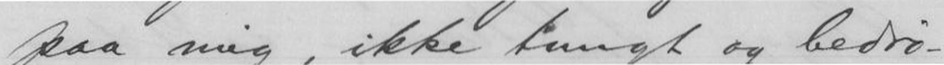paa mig, ikke tungt og bedrø-
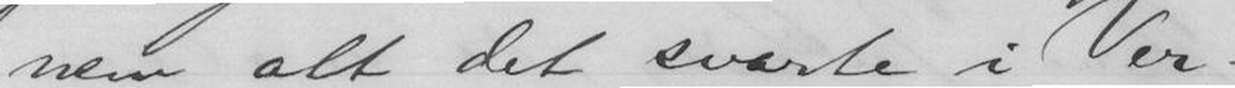nem alt det svarte i Ver-
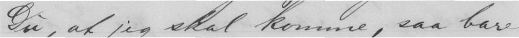Du, at jeg skal komme, saa bare
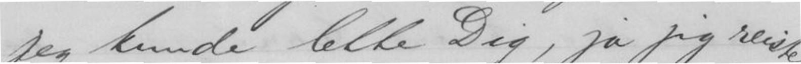jeg kunde lette Dig, ja jeg reiste
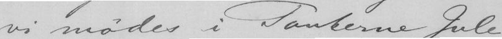vi mødes i Tankerne Jule-
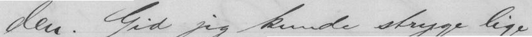den. Gid jeg kunde stryge lige
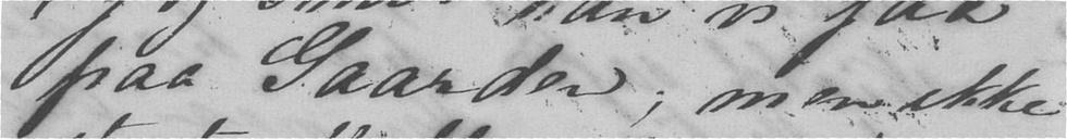paa Gaarden; men ikke
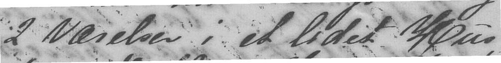2 Værelser i et lidet Hus
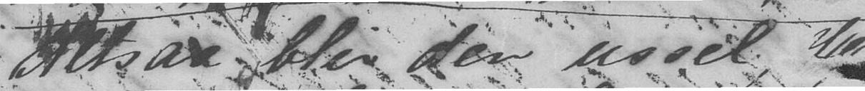Altsaa blir der ussel H
